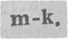m-k.
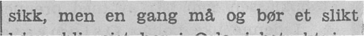sikk, men en gang må og bør et slikt
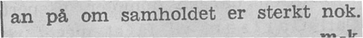an på om samholdet er sterkt nok.
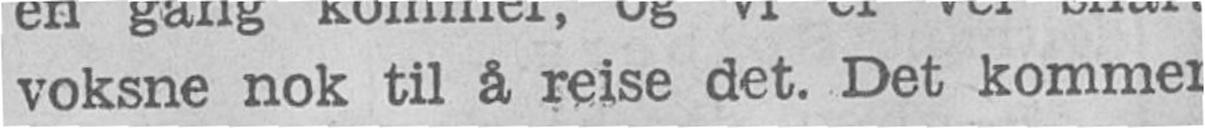voksne nok til å reise det. Det kommer
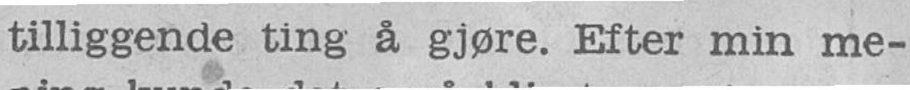tilliggende ting å gjøre. Efter min me-
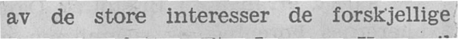av de store interesser de forskjellige
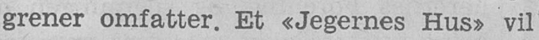grener omfatter. Et "Jegernes Hus" vil
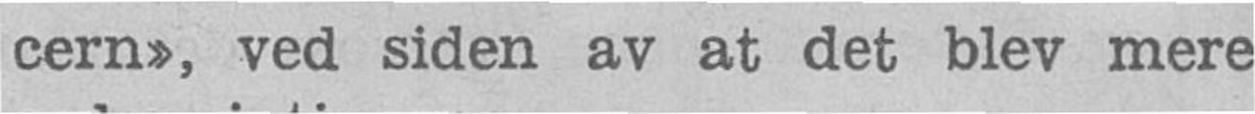cern», ved siden av at det blev mere
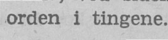orden i tingene.
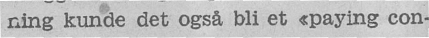ning kunde det også bli et «paying con-
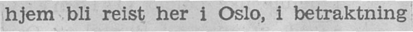hjem bli reist her i Oslo, i betraktning
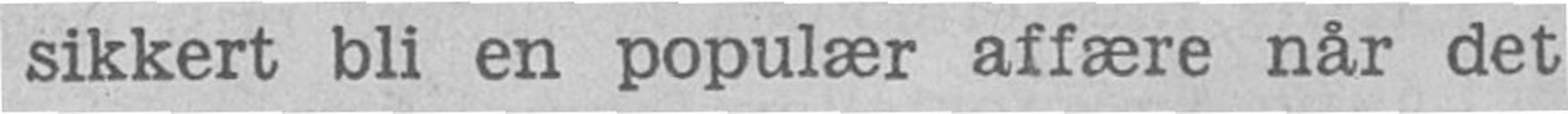sikkert bli en populær affære når det
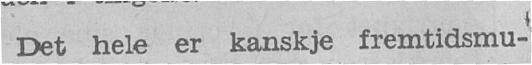Det hele er kanskje fremtidsmu-
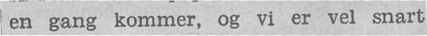en gang kommer, og vi er vel snart
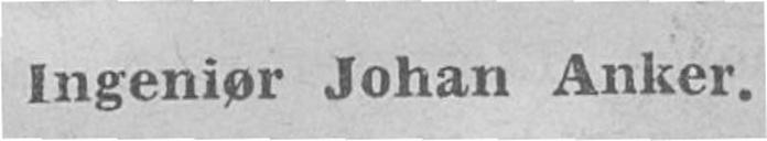Ingeniør Johan Anker.
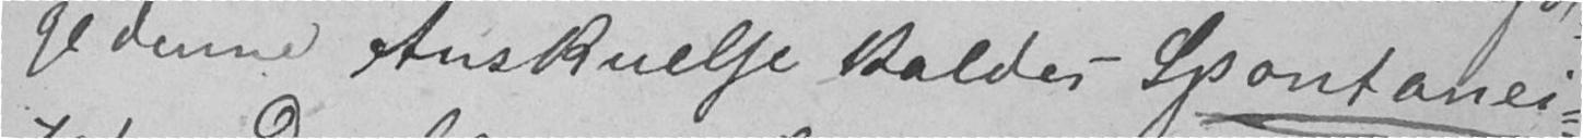ge denne Anskuelse kaldes Spontanei-
